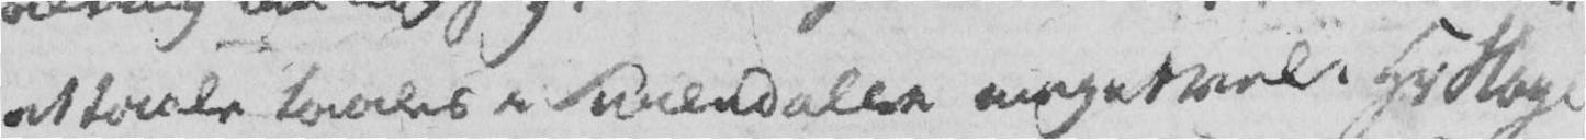at taale taales i Surendalen meget vel. Hr Hage-
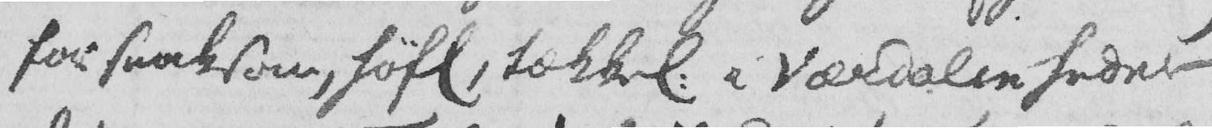for snaksom, høfl, tækkel i Værdalen heder
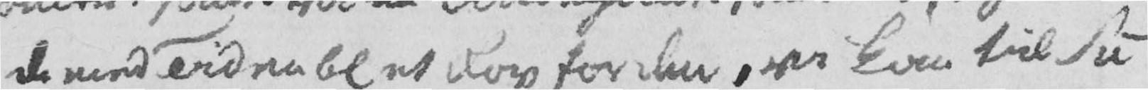de med Tiden bl et Rov for den, vi kom til Su-
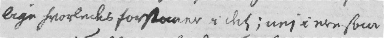lige hvorledes forstaaer i det; nej i ere saa
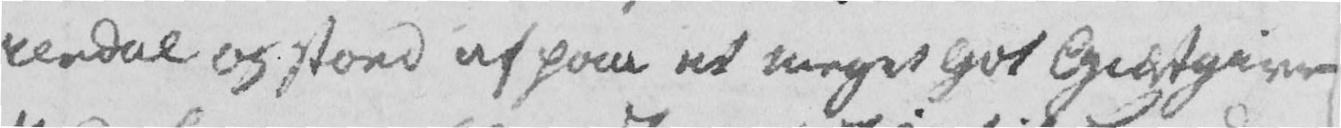rendal og stoed af paa et meget got Giæstgiver-
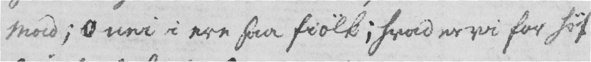Mad; O nei i ere saa fiølk; hvad er vi for høf-
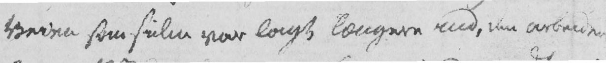Veien som siden var lagt længere ind, den arbeider
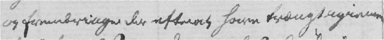og frembringer der efterat have trængt igiennem
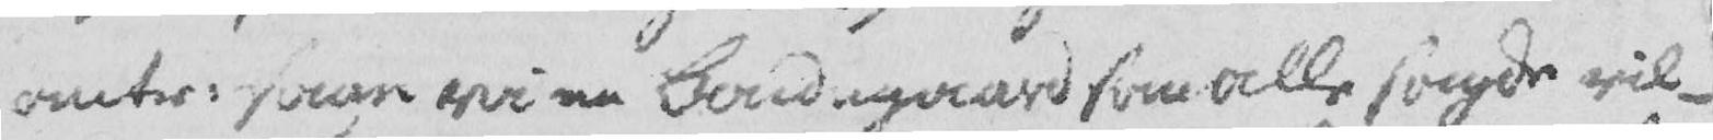omtr. saa vi en Bondegaard som alle sagde vil-
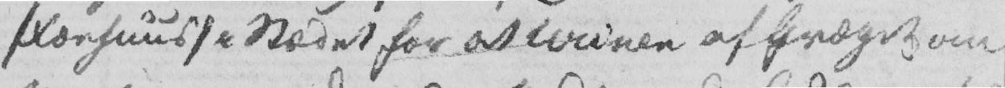/ Fæehuus / i stædet for at Urinen af Qvæget om
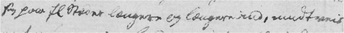sig paa fl Stæder længere og længere ind, midtveis
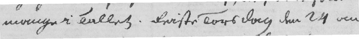mange i Tallet. Reiste Torsdag den 24 om
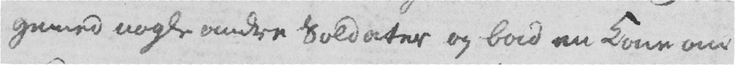gemed nogle andre Soldater og bad en Kone om
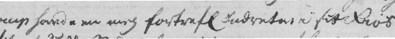rup havde en meg fortrefl Indretn. i sit Fiøs
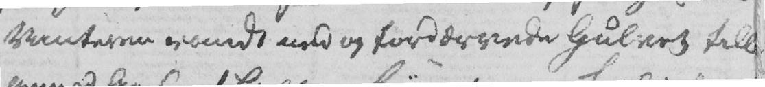Vinteren randt ned og fordærvede Gulvet tilli-
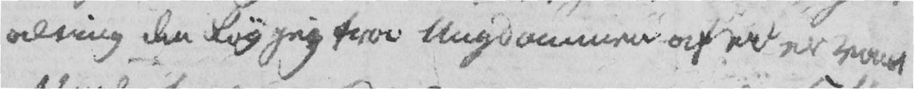alting den Røg jeg troe Ungdommen altid er vant
